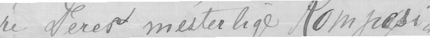re Deres mesterlige Komposi-
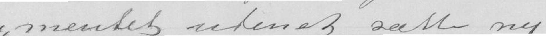mentet uten at sætte ny
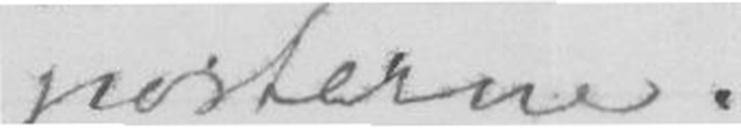porterne.
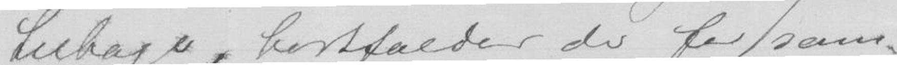tilbage, bortfalder de for sam-
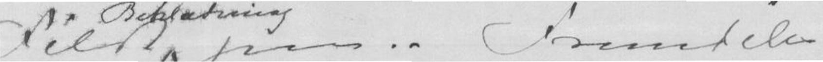Fildt paa. Fremdeles
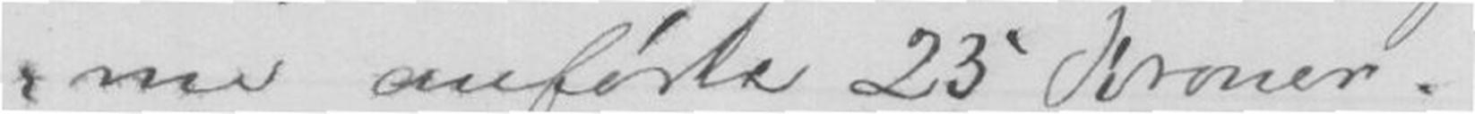me anførte 25 Kroner. -
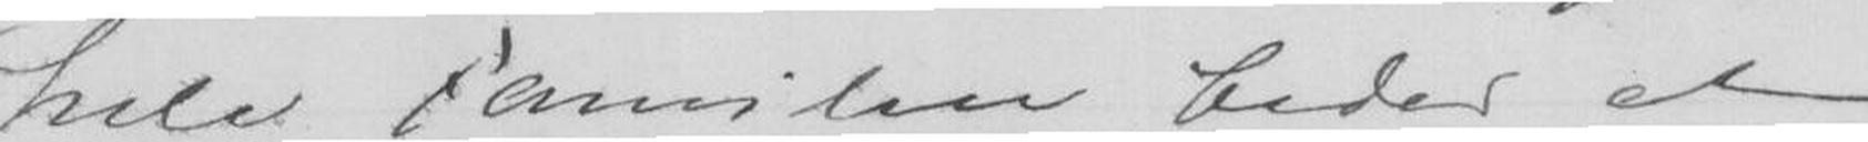hele Familien beder at
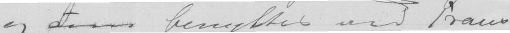og som benyttes ved Trans-
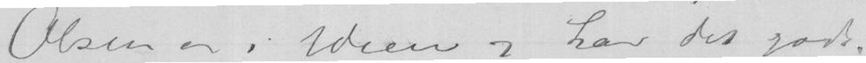Olsen er i Wien og har det godt,
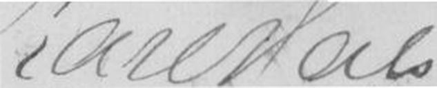Karl Hals
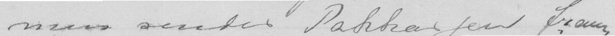men sendes Pakkassen fram og
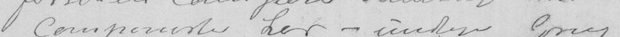componister her - undtage Grieg
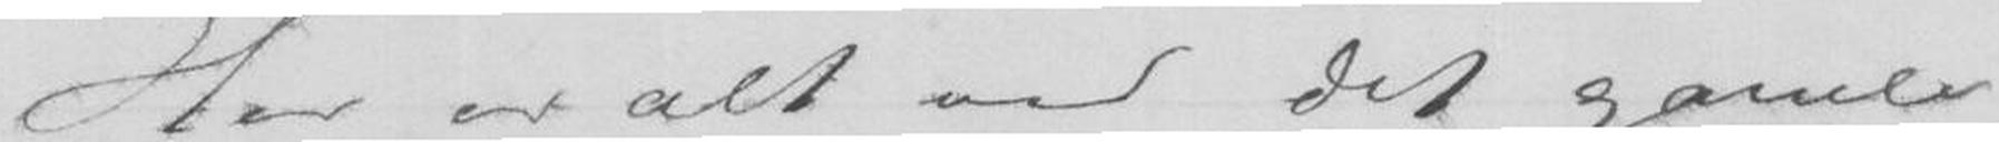Her er alt ved det gamle
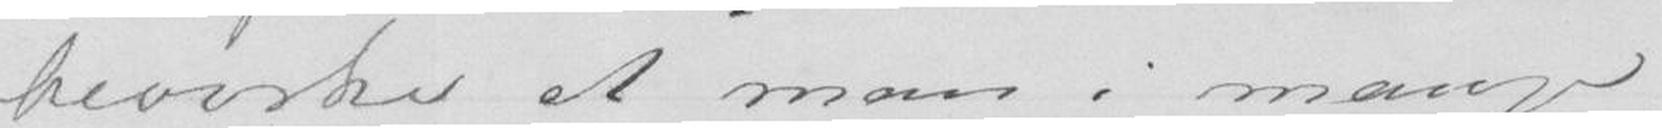bevirke at man i mange
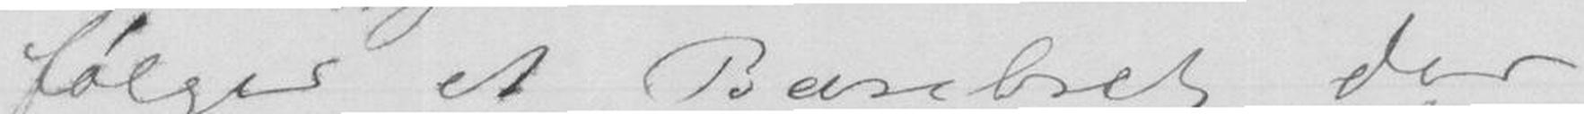følger et Bærebret der
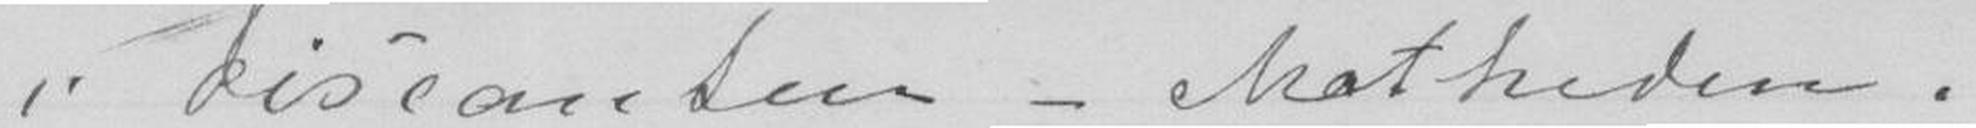i Discanten - Matheden i
